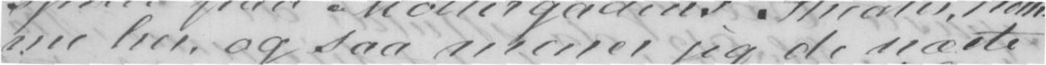me her, og saa mener jeg de næste
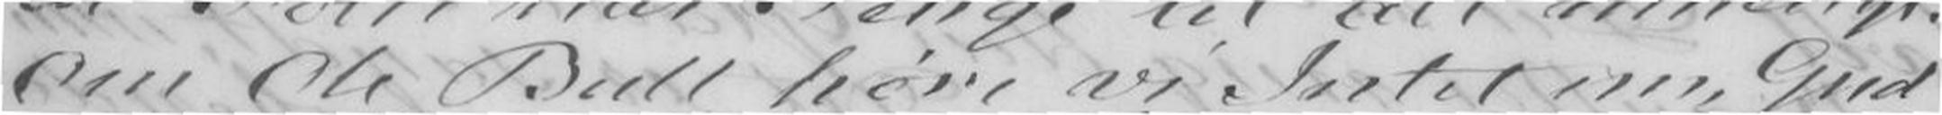Om Ole Bull høre vi Intet nu, Gud
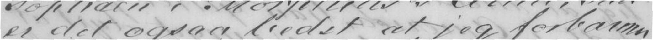er det ogsaa bedst at jeg forbarmer
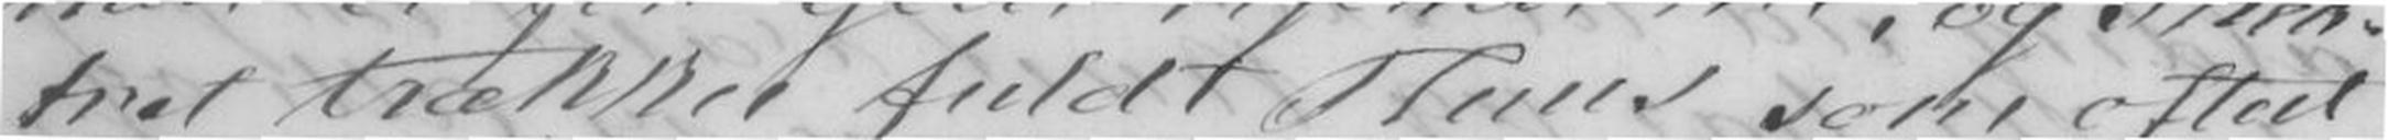tret trækker fuldt Huus som oftest
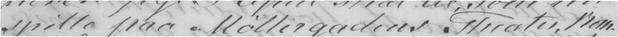spiller paa Møllergadens Theater, kom-
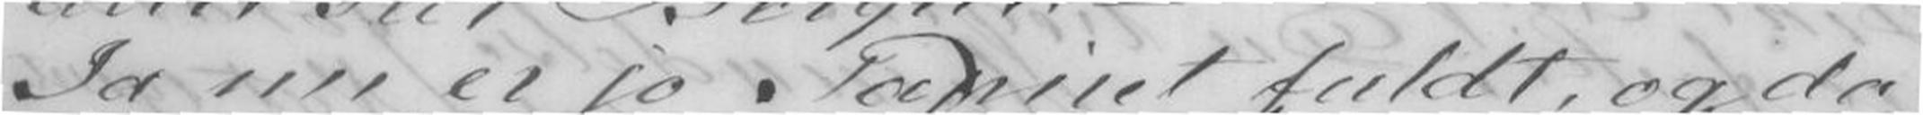Ja nu er jo Papiret fuldt, og da
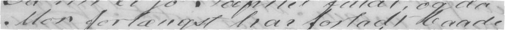Mor forlængst har forladt baade
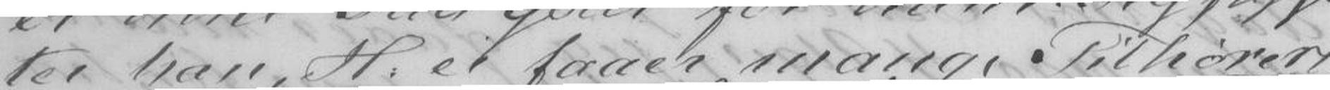ter han, H. ei faaer mange Tilhørere,
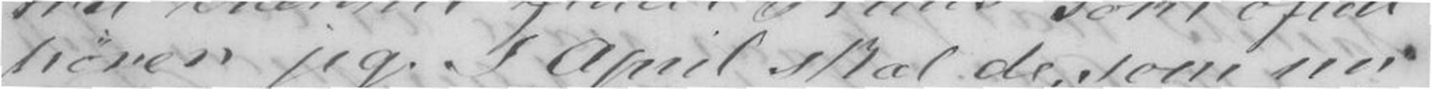hører jeg. I April skal de, som nu
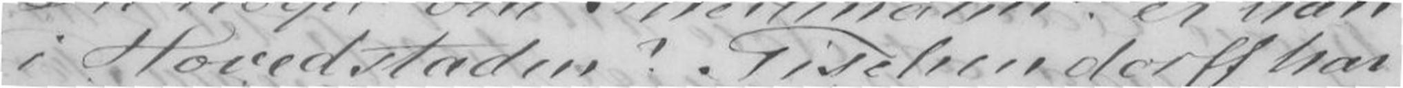i Hovedstaden? Tischendorff har
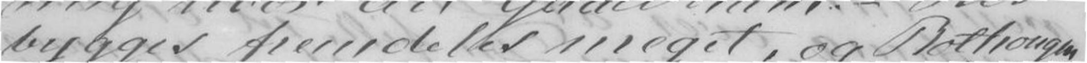bygges fremdeles meget, og Rothougen
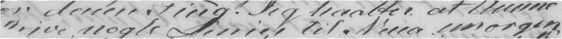ive nogle Linier til Nina imorgen
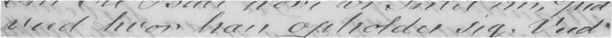veed hvor han opholder sig. Veed
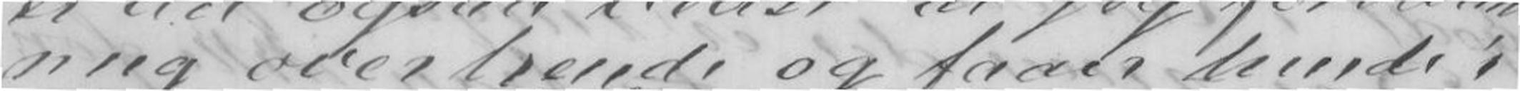mig over hende og faaer hende i
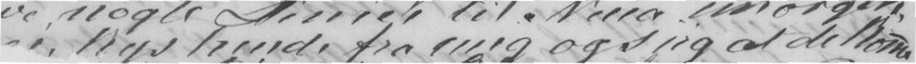kys hende fra mig og siig at de ko'me
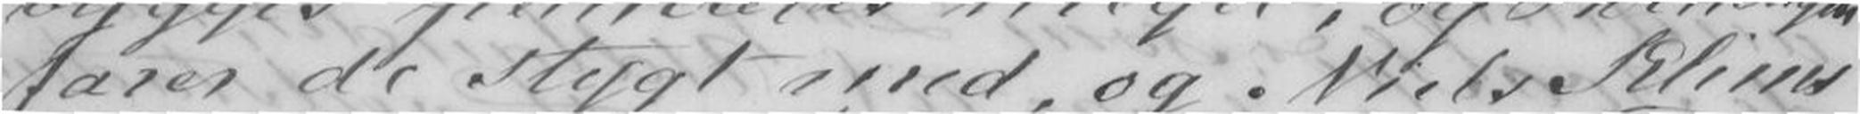farer de stygt med, og Niels Klims
